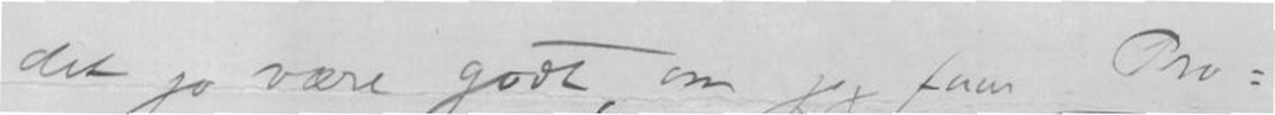det jo være godt, om jeg faar Pro-
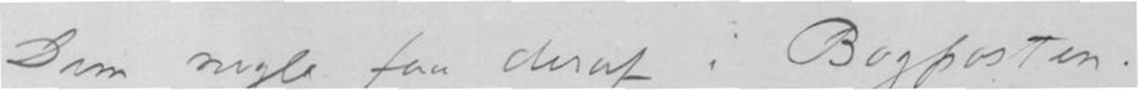Dem nogle faa deraf i Bogposten. -
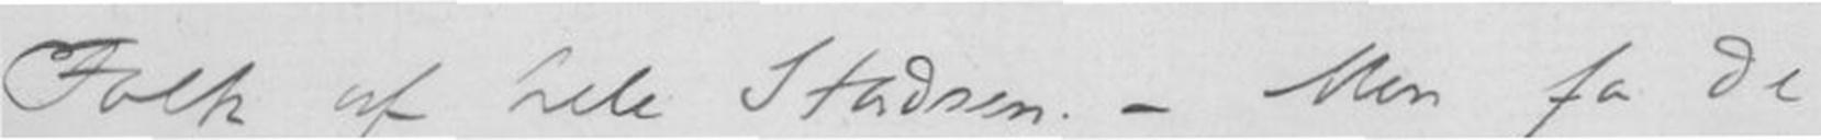Folk af hele Stadsen. - Men for De
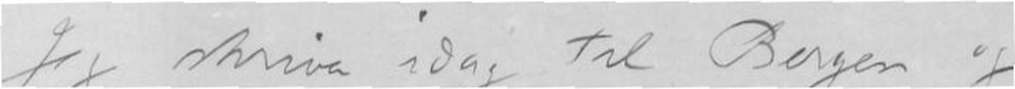Jeg skriver idag til Bergen og
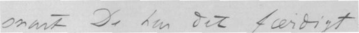snart De har det færdigt.
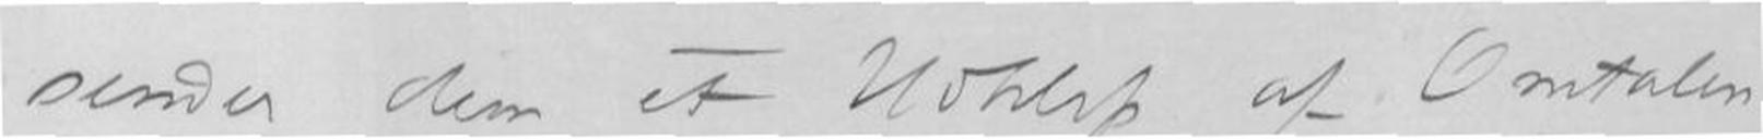sender dem et Udklip af Omtalen
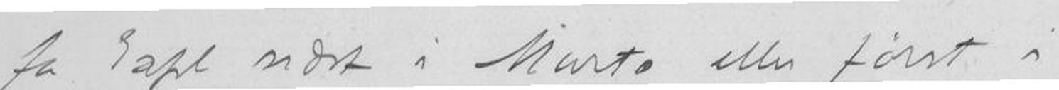for Expl. sidst i Marts eller først i
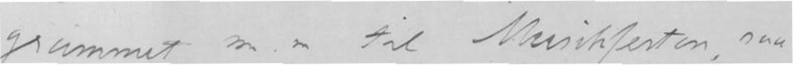grammet m.m. til Musikfesten, saa
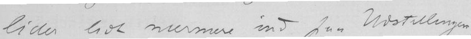lider lidt nærmere ind paa Udstillingen,
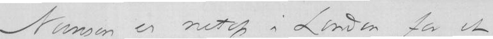Nansen er netop i London for et
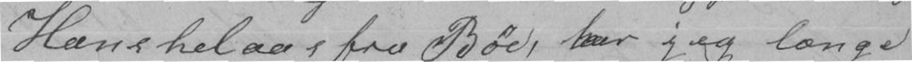Hans helaas fra Bøe, har jeg længe
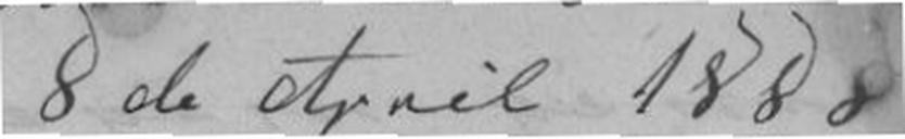8 de April 1888
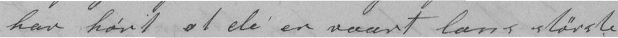har hørt at de er vaart lans største
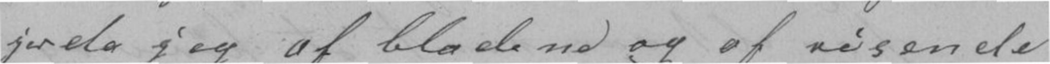jer da jeg af bladene og af visende
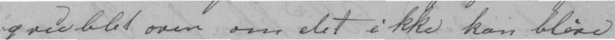grublet over om det ikke kan blive
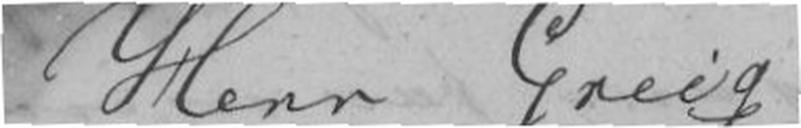Herr Greig
